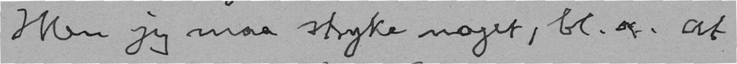Men jeg maa stryke noget, bl. a. at
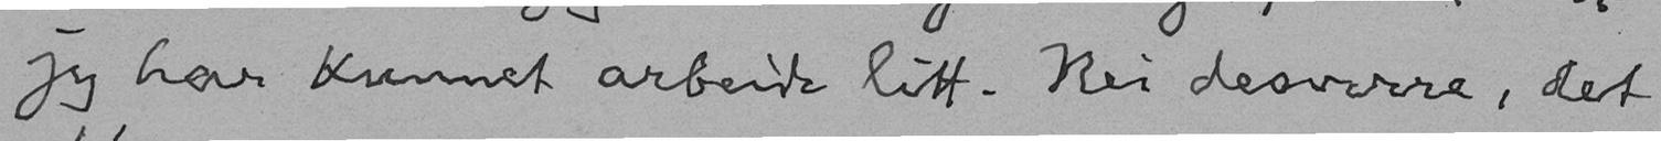jeg har kunnet arbeide litt. Nei desverre, det
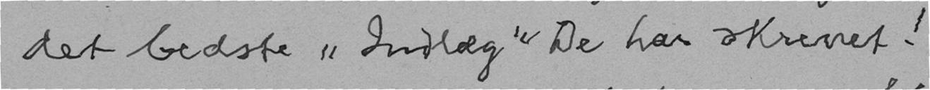det bedste "Indlæg" De har skrevet!
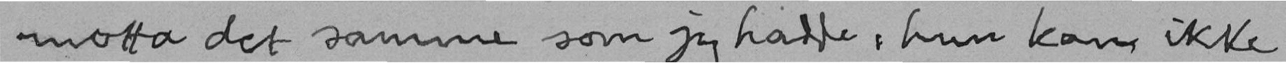motta det samme som jeg hadde, hun kan ikke
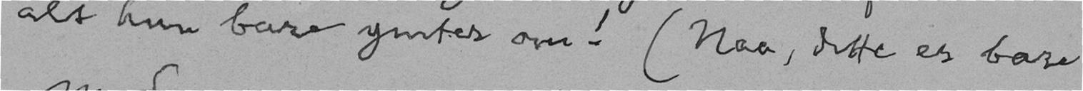alt hun bare ymter om! (Naa, dette er bare
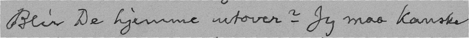Blir De hjemme utover? Jeg maa kanske
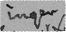ingen
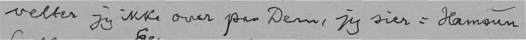velter jeg ikke over paa Dem, jeg sier: Hamsun
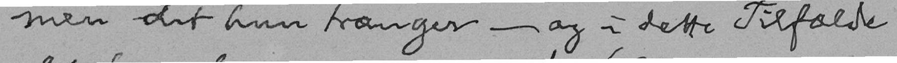men det hun trænger - og i dette Tilfælde
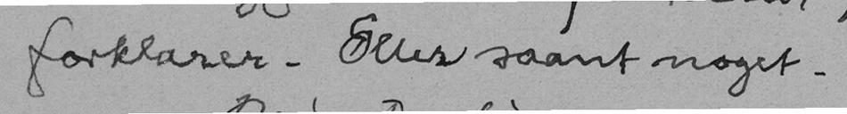forklarer. Eller saant noget.
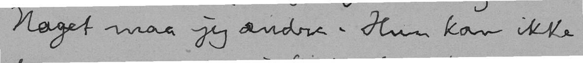Noget maa jeg ændre. Hun kan ikke
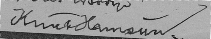Knut Hamsun.
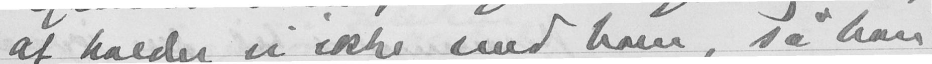at holder vi ikke med ham, så han
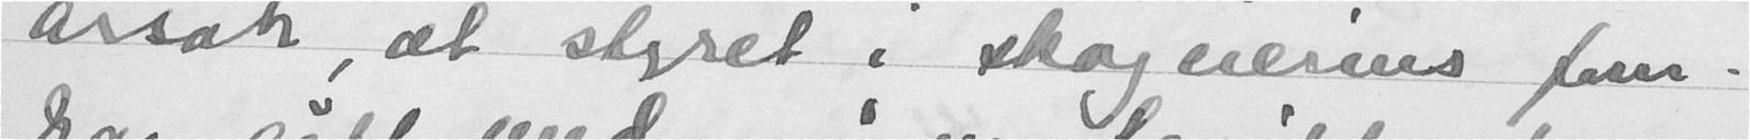årsak, at styret i skogeieres favn
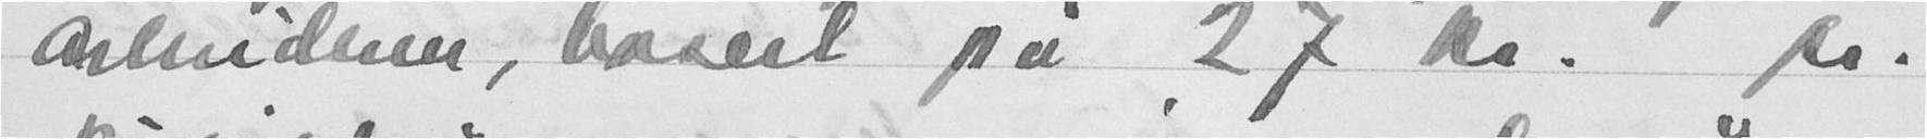arbeiderne, basert på 27 kr. pr.
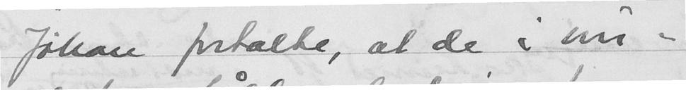Johan fortalte, at de i vin-
